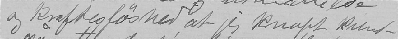og kraftesløshed at jeg knapt kunde
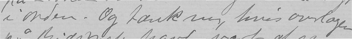i orden. Og tænk nu, hvis overlægen
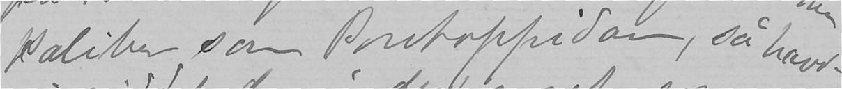kaliber som Pontoppidan, så havde
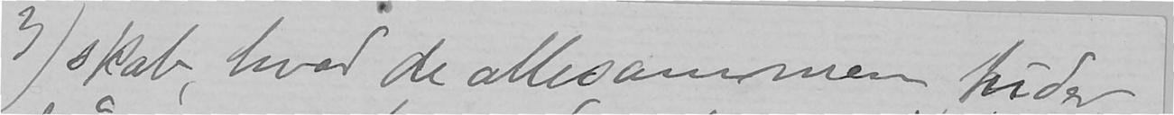3) skab, hvad de allesammen bider
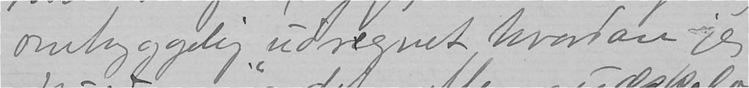omhyggelig udregnet hvordan jeg
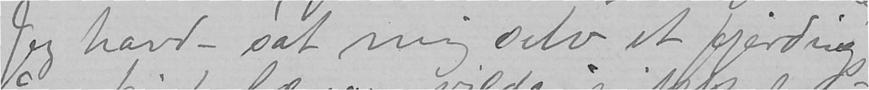Jeg havde sat mig selv et fjerdings-
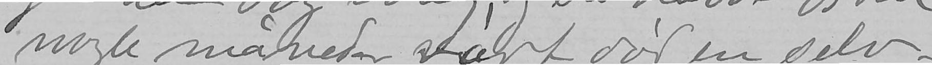nogle måneder vært død en selv-
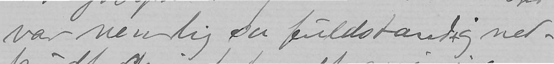var nemlig nu fuldstændig ned-
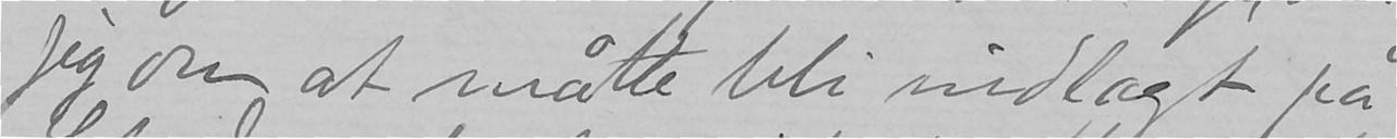jeg om at måtte bli indlagt på
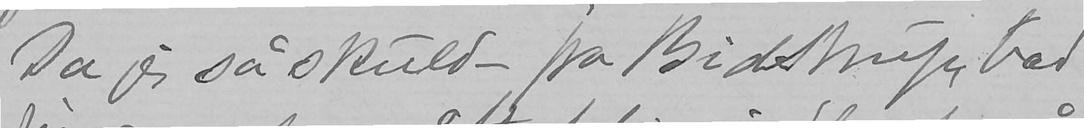Da jeg så skulde på Bidstrup, bad
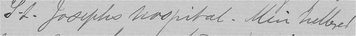St. Josephs hospital. Min helbred
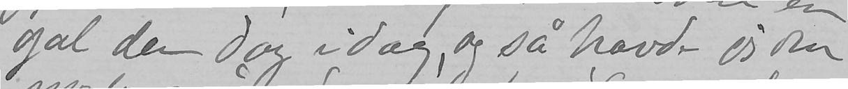gal den dag i dag, og så havde jeg om
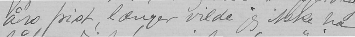års frist, længer vilde jeg ikke ha
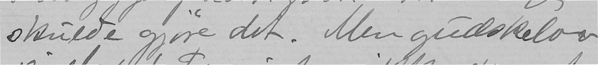skulde gjøre det. Men gudskelov
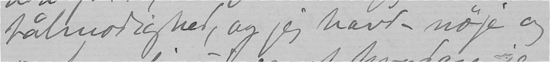tålmodighed, og jeg havde nøje og
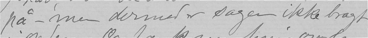på - men dermed er sagen ikke bragt
